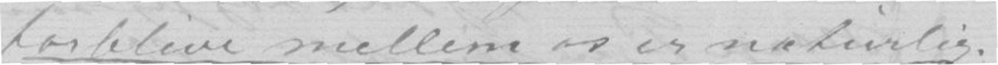forblive mellem os er naturlig.
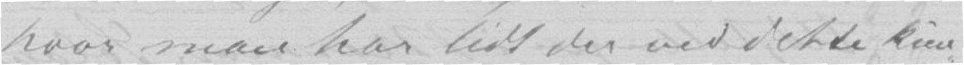hvor man har lidt der ved dette kun-
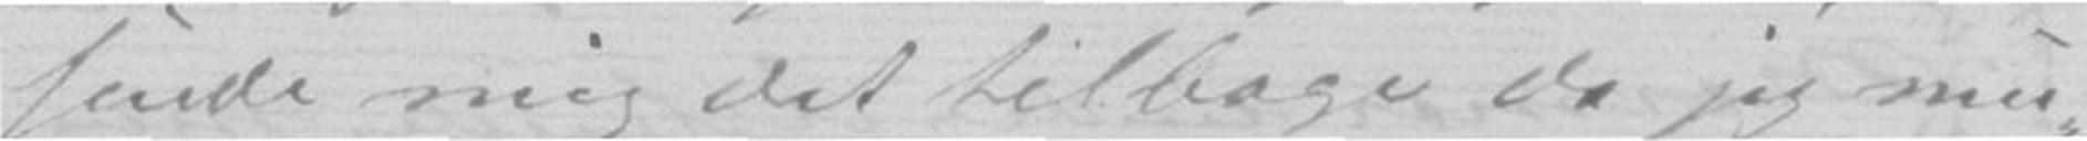sende mig det tilbage da jeg mu-
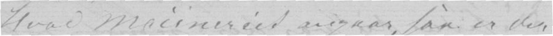Hvad Macineriet angaar, saa er der
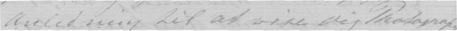Anledning til at vise dig Photografi
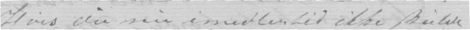Hvis du nu imidlertid ikke skulde
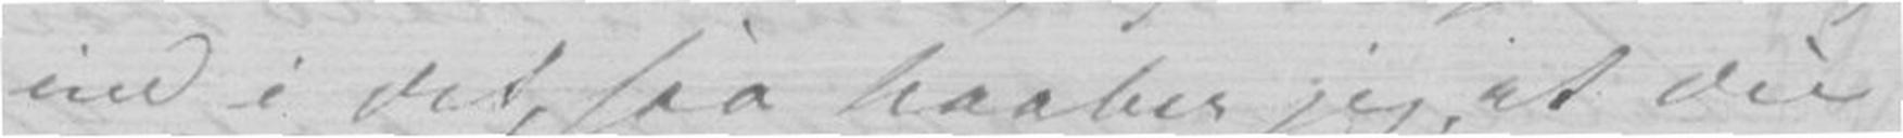ind i det, saa haaber jeg, at du
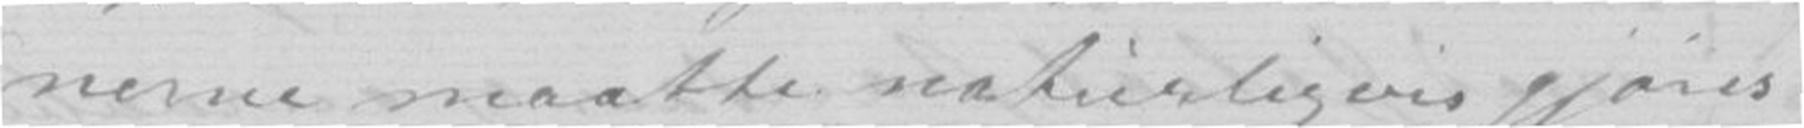erne maatte naturligvis gjøres
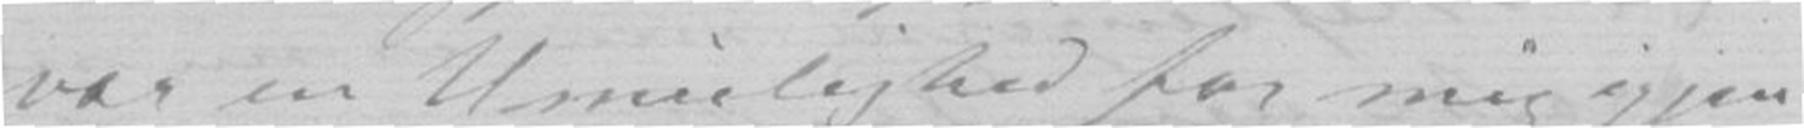var en Umulighed for mig igjen
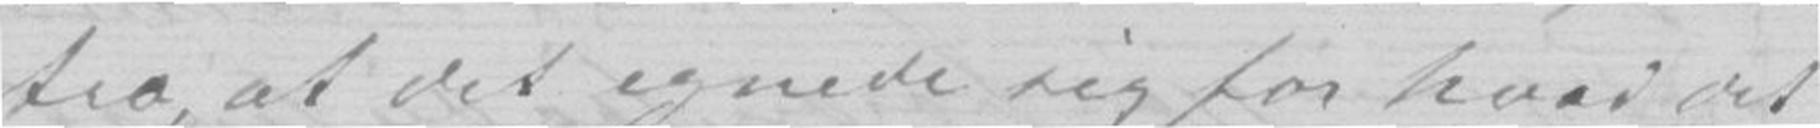tro, at det egnede sig for hvad det
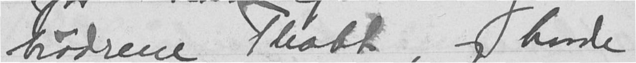brødrene Thott, og havde
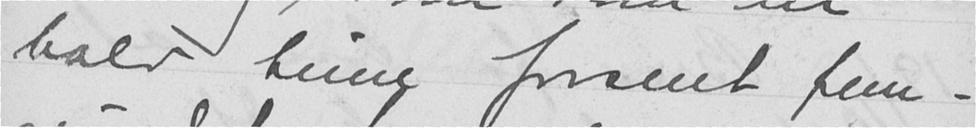halv time forsent frem -
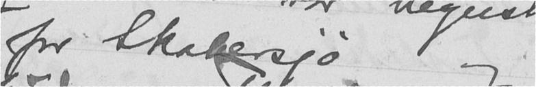for Skabersjø og
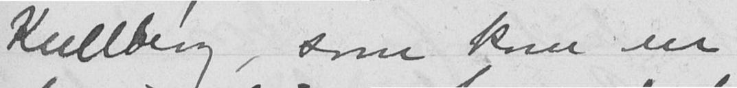Kullberg, som kom en
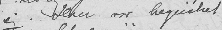sig. Han var begeistret
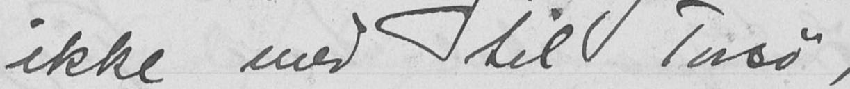ikke med til Torsø,
